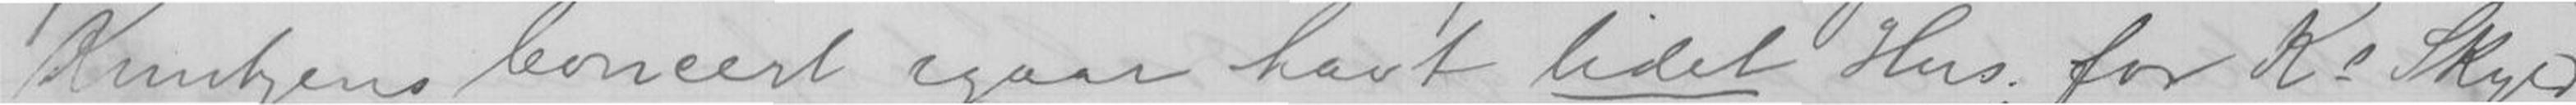Knutzens Concert igaar havt lidet Hus; for Ks Skyld
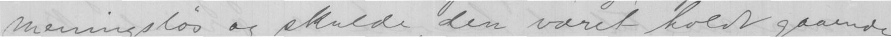meningsløs og skulde den været holdt gaaende
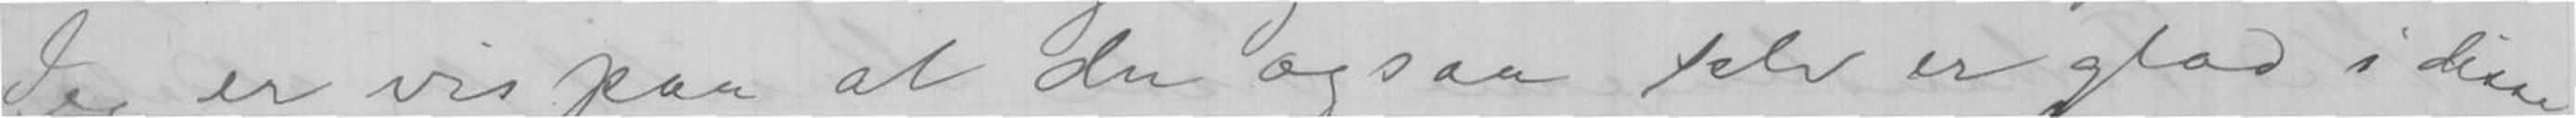Jeg er vis paa at du ogsaa selv er glad i disse
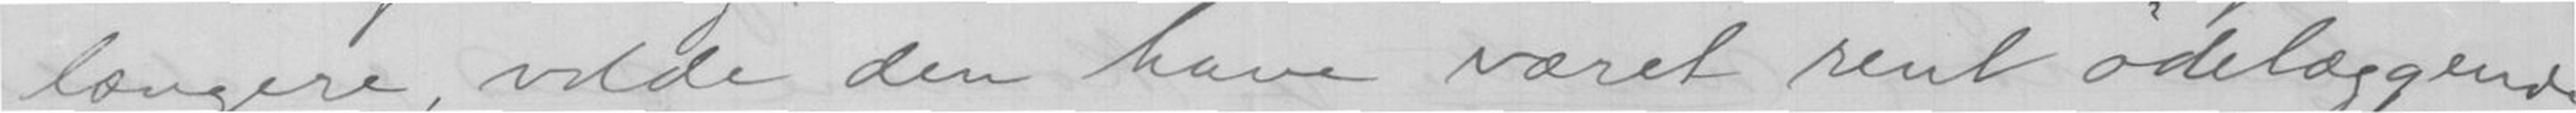længere, vilde den have været rent ødelæggende
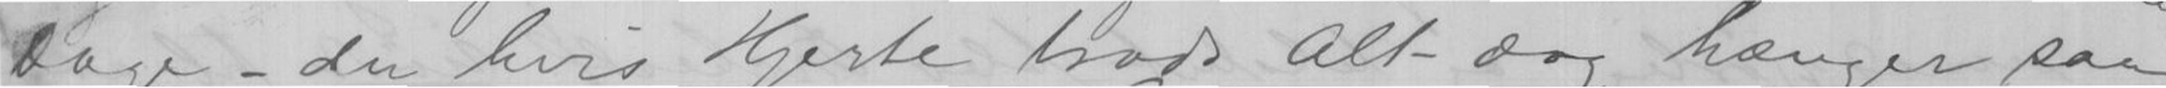Dage - du hvis Hjerte trods Alt dog hænger saa
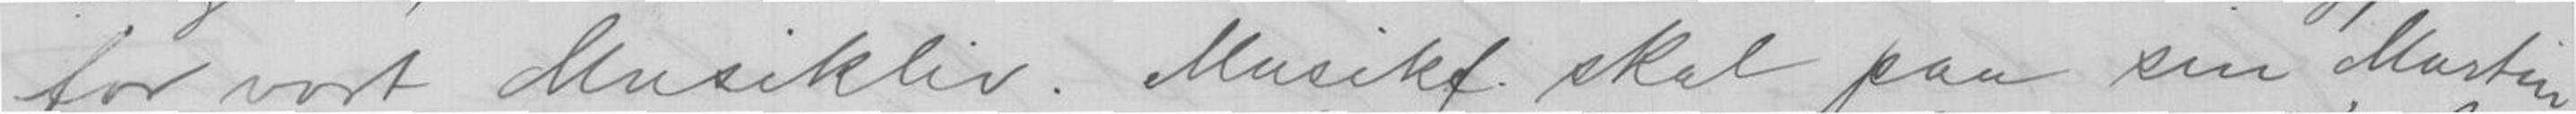for vort Musikliv, Misikf. skal paa sin Martin
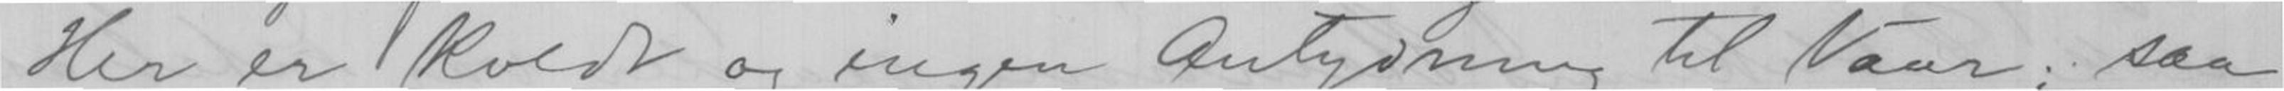Her er koldt og ingen Antydning til Vaar; saa
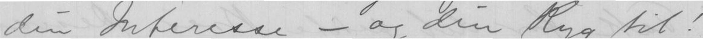din Interresse - og din Rygg til!
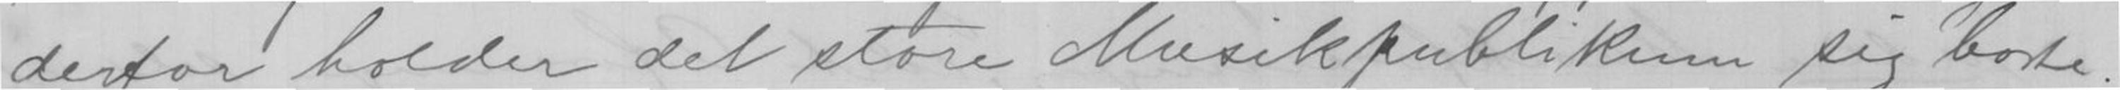derfor holder det store Musikpublikum sig borte.
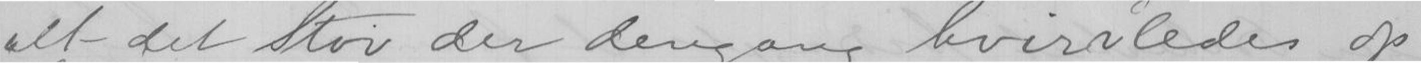alt - det Støv der dengang hvirvledes op
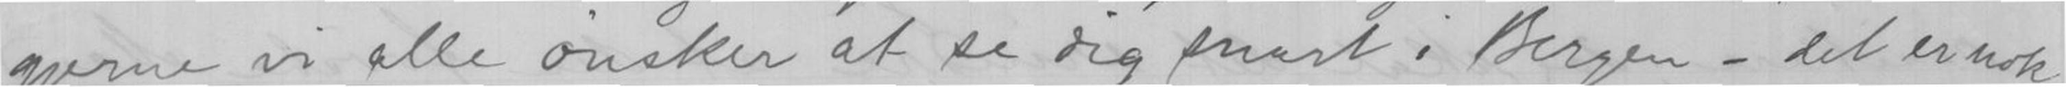gjerne vi alle ønsker at se dig snart i Bergen - det er nok
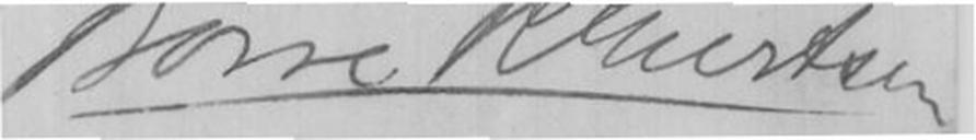Børre R Giertsen
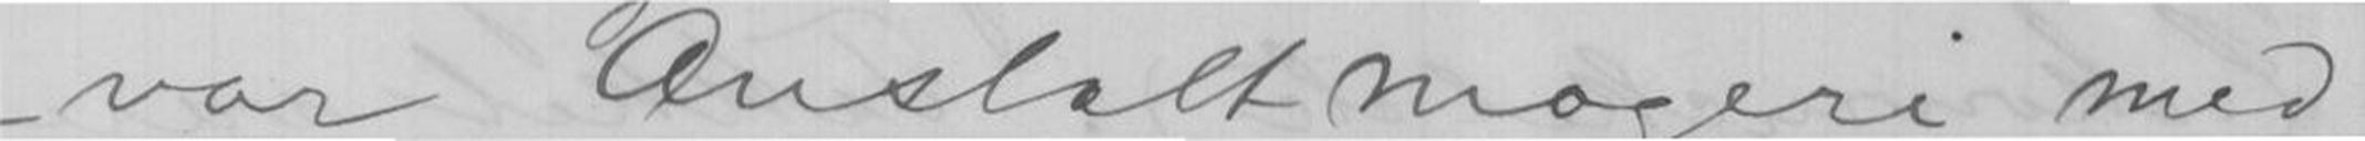var Anstaltmageri med
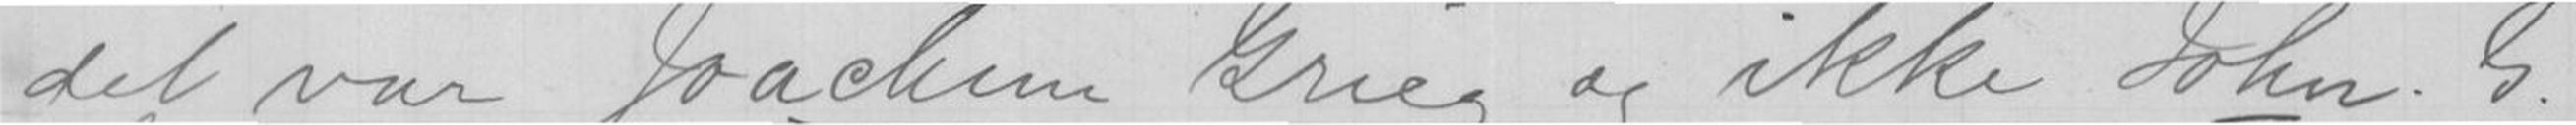det var Ooachim Grieg og ikke John G.
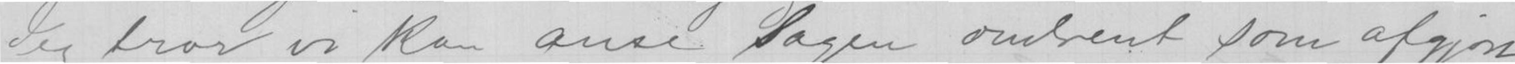Jeg tror vi kan anse Sagen omtrent som afgjort
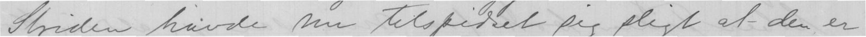Striden havde nu tilspidset sig sligt at den er
